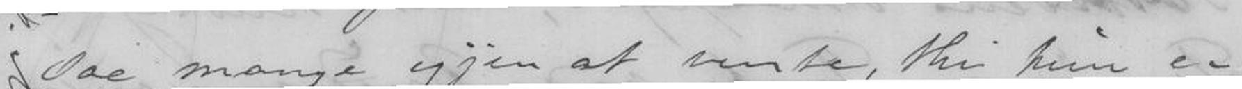saa mange igjen at vente, thi hun er
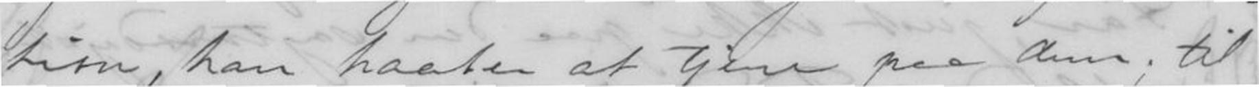tion, han haaber at tjene paa dem; til
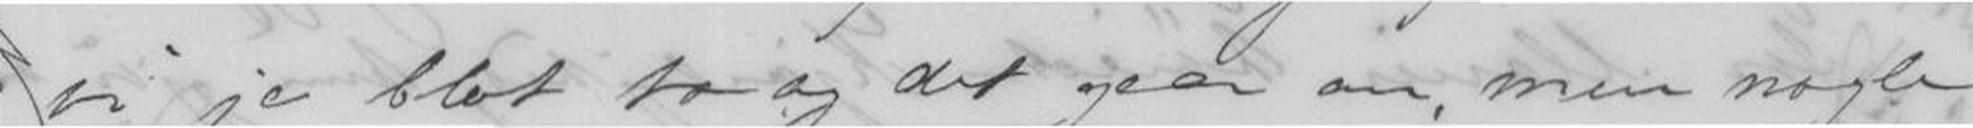vi jo blot to og det gaar an, men nogle
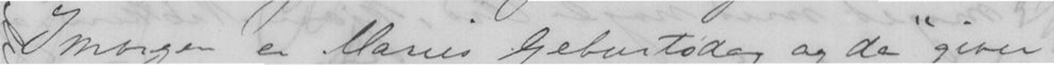Imorgen er Maries Geburtsdag og de giver
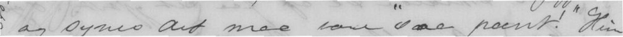og synes det maa være "saa pænt!" Hun
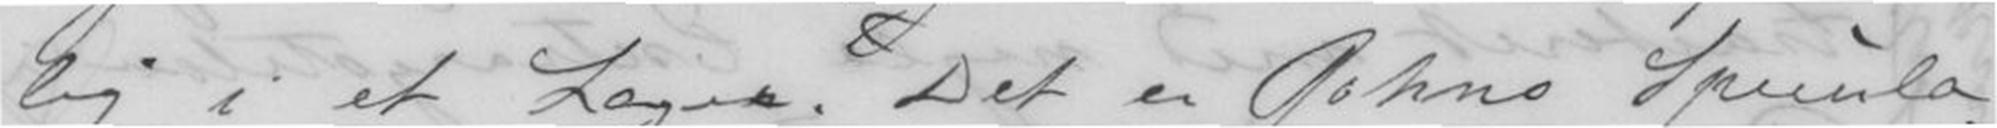lig i et Lager. Det er Johns Spunla-
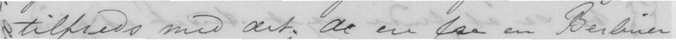tilfreds med det; de ere fra en Berliner
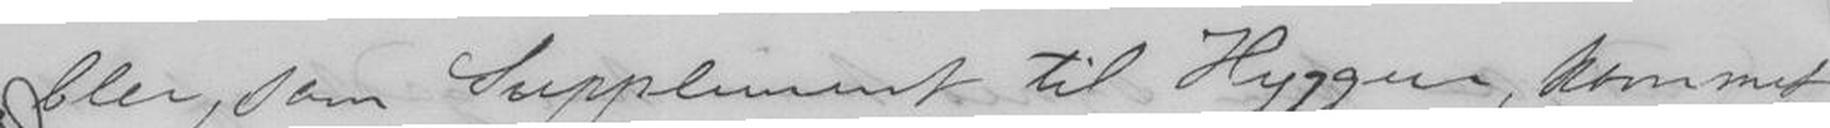blev, som Supplement til Hyggen, kommet
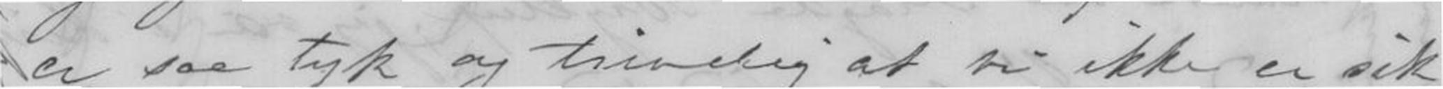er saa tyk og trivelig at vi ikke er sik-
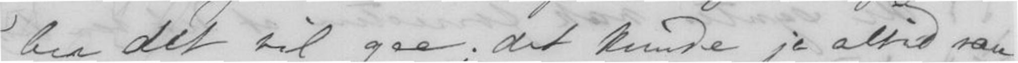ber det vil gaa; det kunde jo altid være
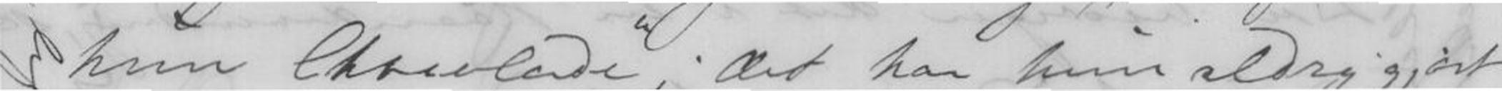hun Chocolade; det har hun aldrig gjort
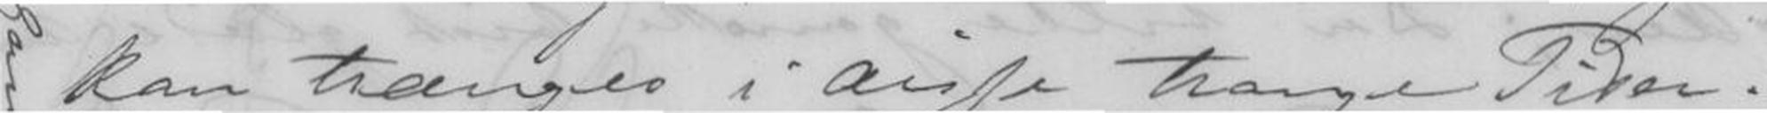kan trænges i disse trange Tider.
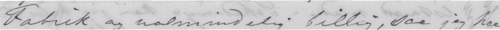Fabrik og ualmindelign billig, saa jeg haa-
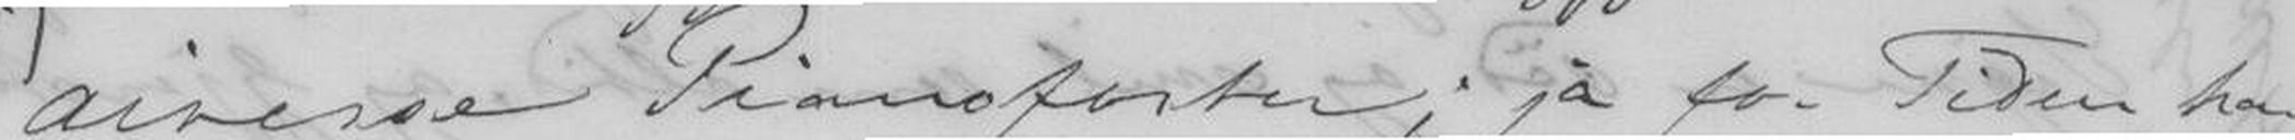diverse Pianoforter; ja for Tiden har
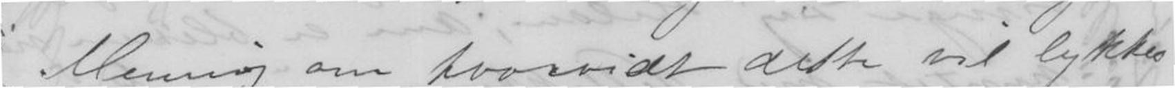Mening om hvorvidt dette vil lykkes
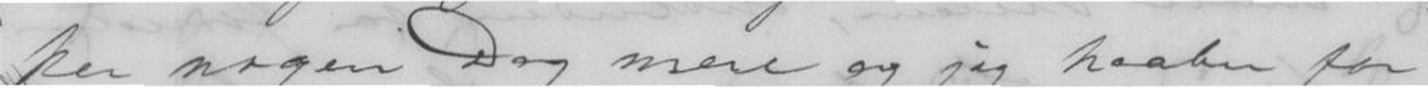ker nogen Dag mere og jeg haaber for
